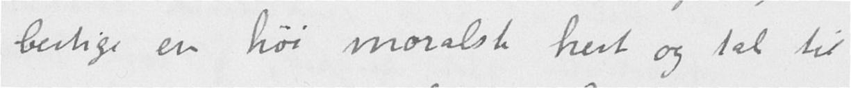bestige en høi moralsk hest og tale til
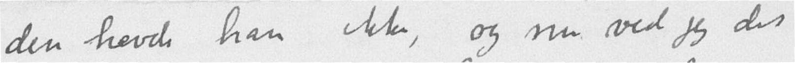den havde han ikke, og nu ved jeg det
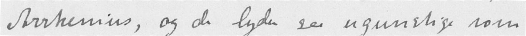Arrhenius, og de lyder saa ugunstige som
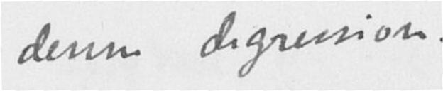denne digression.
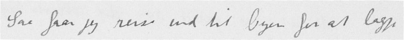Saa faar jeg reise ind til byen for at lægge
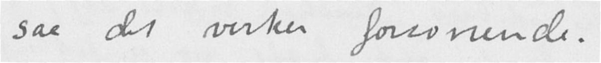saa det virker forsonende.
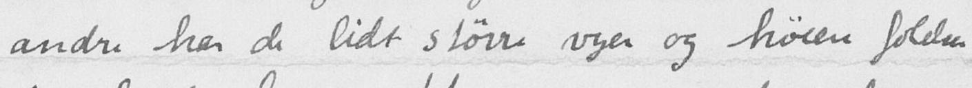andre har de lidt større vyer og høiere følelser
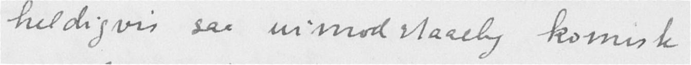heldigvis saa uimodstaaelig komisk
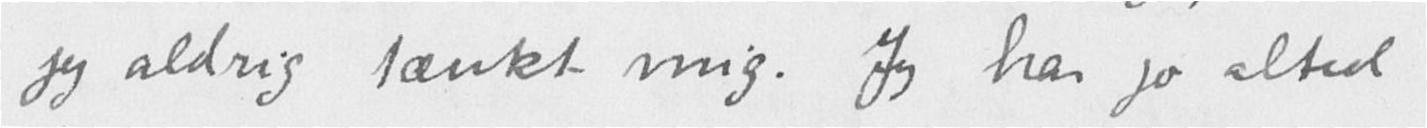jeg aldrig tænkt mig. Jeg har jo altid
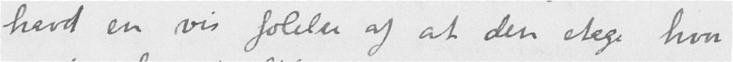havd en vis følelse af at den stage hvor
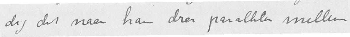dig det naar han drar paralleler mellem
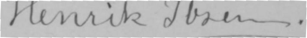Henrik Ibsen.
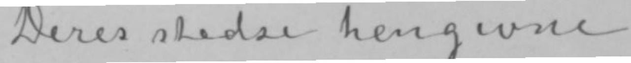Deres stedse hengivne
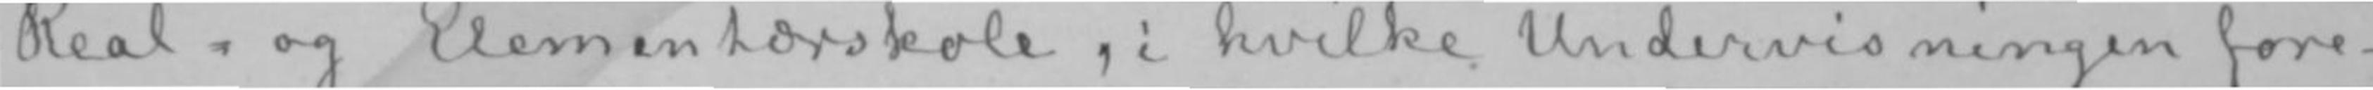Real- og Elementærskole, i hvilke Undervisningen fore-
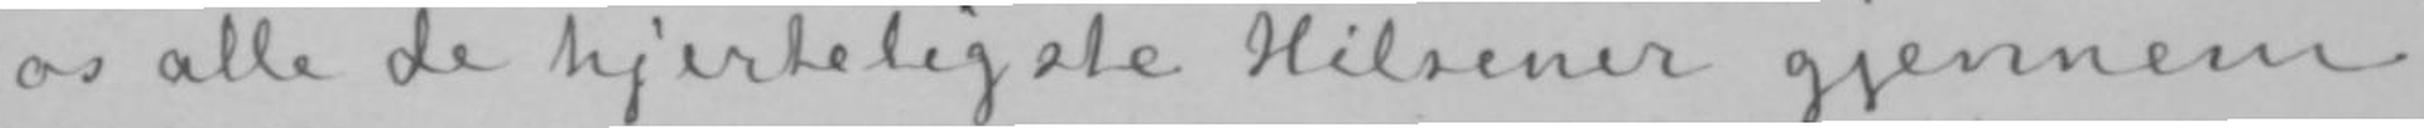os alle de hjerteligste Hilsener gjennem
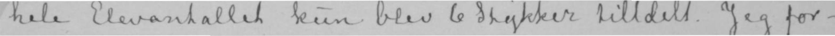hele Elevantallet kun blev 6 Stykker tilldelt. Jeg for-
